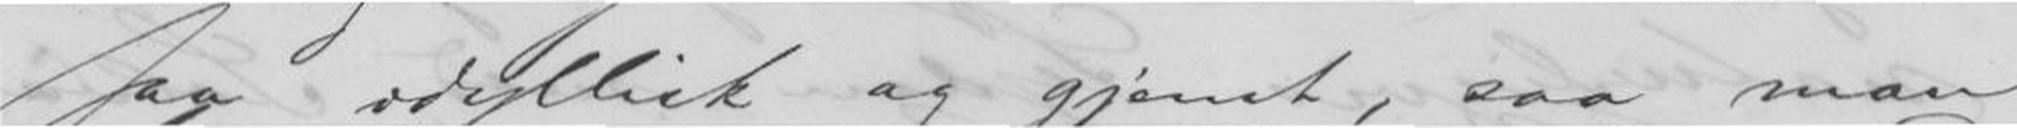saa idyllisk og gjemt, saa man
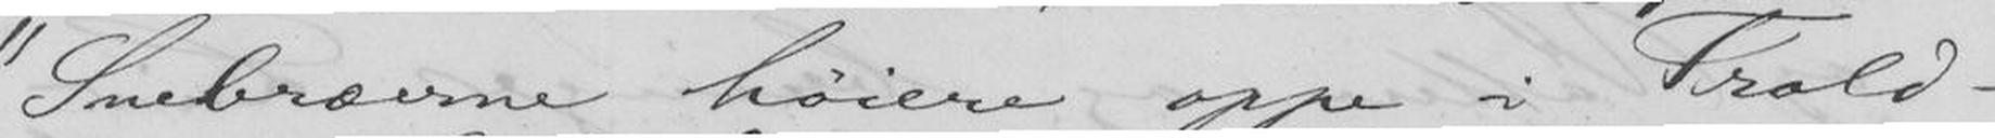"Snebræerne høiere oppe i Trold-
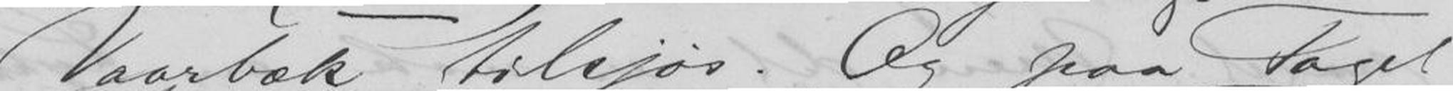Vaarbæk tilsjøs. Og paa Taget
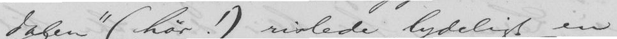dalen" (hør!) rislede tydeligt en
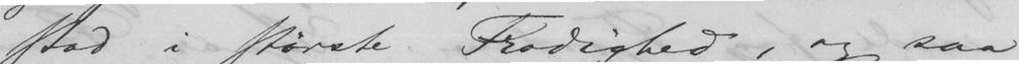stod i største Frodighed, og saa
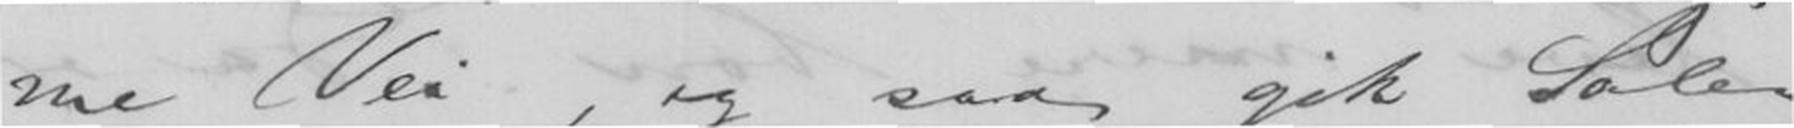me Vei, og saa gik Solen,
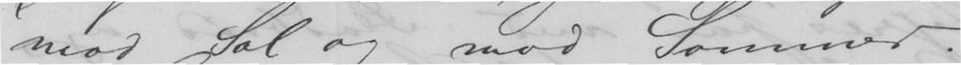mod Sol og mod Sommer.
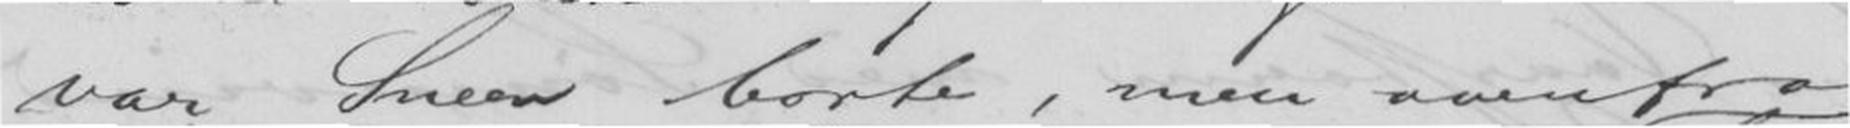var Sneen borte, men ovenfra
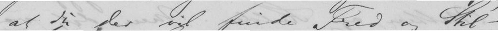at Du der vil finde Fred og Stil-
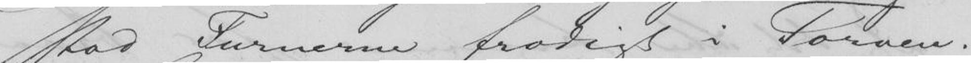stod Furuerne frodigt i Torven.
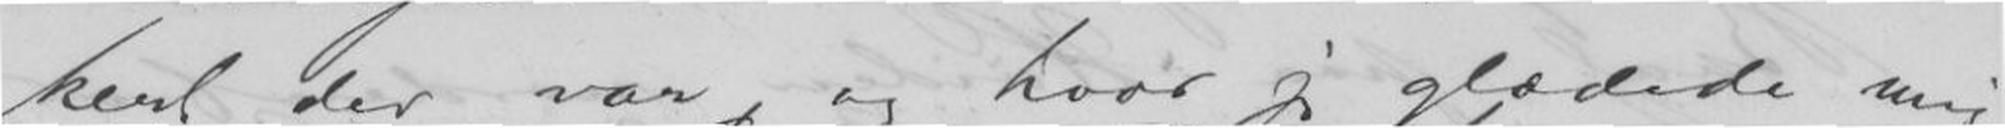kert der var, og hvor jeg glædede mig
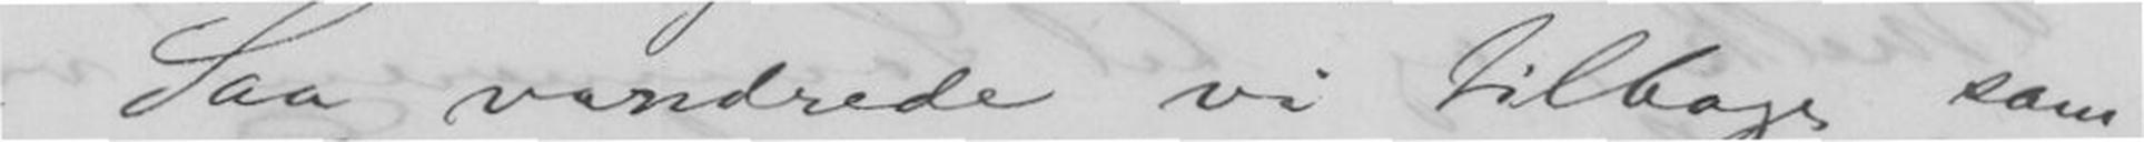Saa vandrede vi tilbage sam-
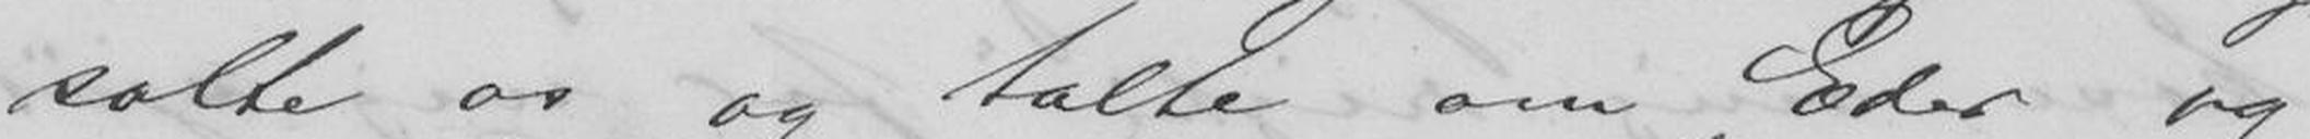satte os og talte om Eder og
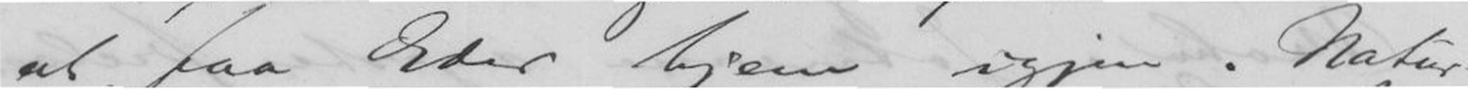at faa Eder hjem igjen. Natur-
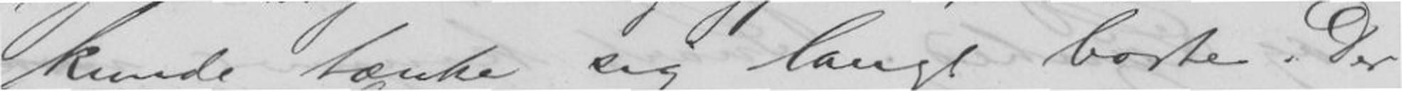kunde tænke sig langt borte. Der
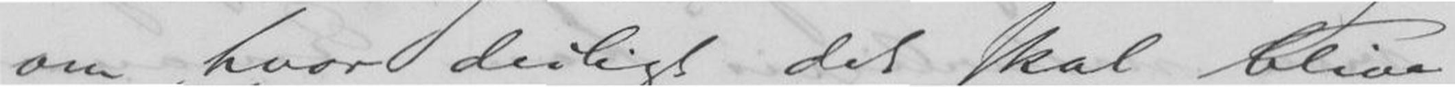om, hvor deilig det skal blive
